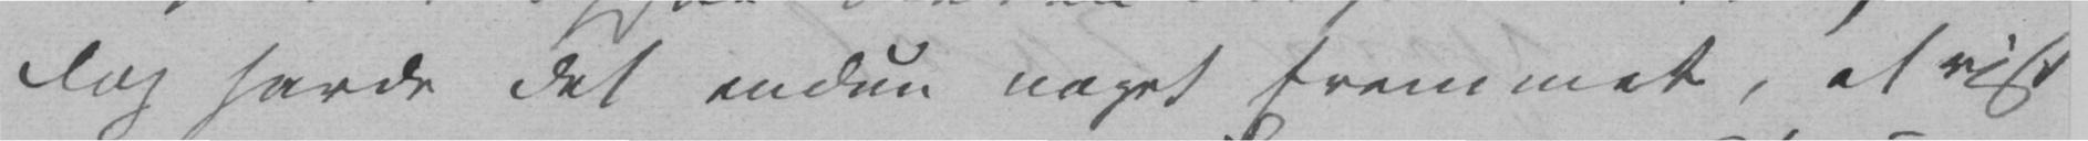dog havde det endnu noget fremmet, et vist
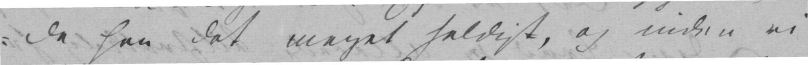-de han det meget heldigt, og inden vi
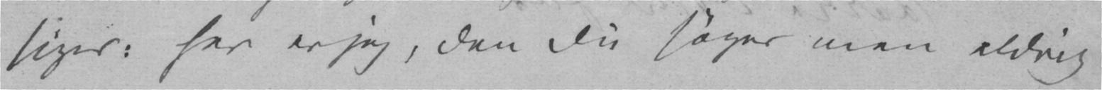siger: her er jeg, den Du søger men aldrig
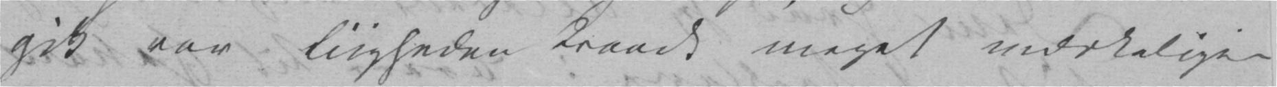gik var Liigheden traadt meget mærkeligere
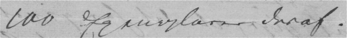100 Exemplarer deraf.
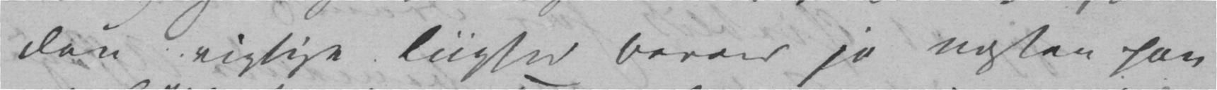den rigtige Liighed beroer jo næsten paa
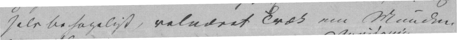selvbehageligt, velnæret Træk om Munden
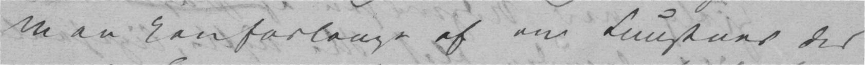man kan forlange af en Kunstner der
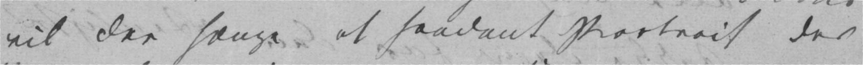vil der hænge et saadant Portrait der
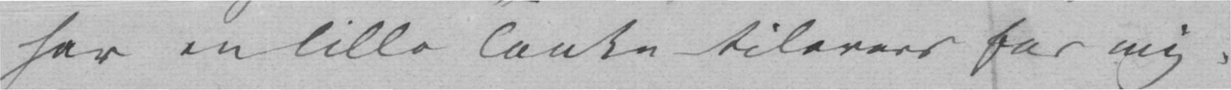har en lille Tanke tilovers for mig.
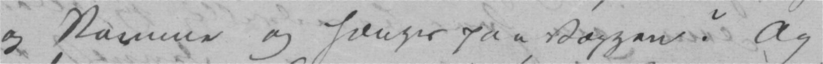og Ramme og hænges paa Væggen? Og
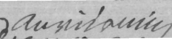Anviisning
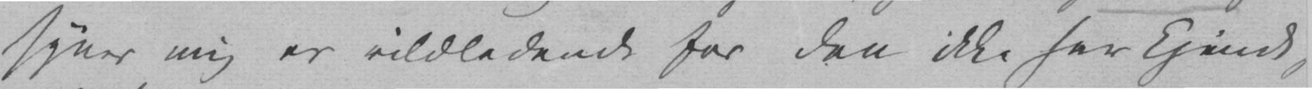synes mig er vildledende for den ikke har kjendt,
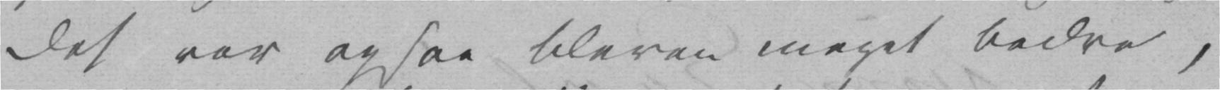det var ogsaa bleven meget bedre,
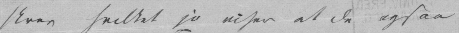skrev hvilket jo viser at De ogsaa
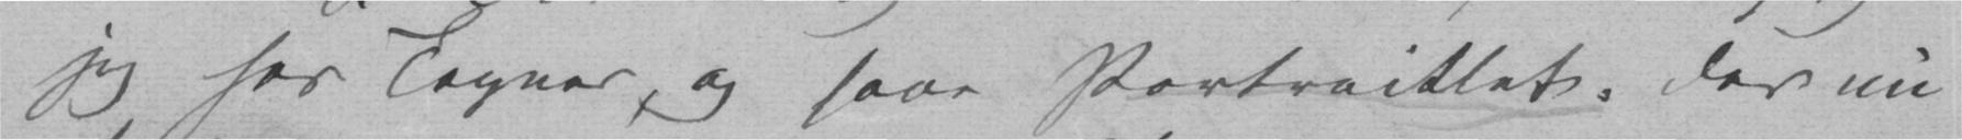jeg hos Tegner, og saae Portraittet, der nu
